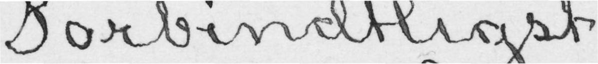Forbindtligst
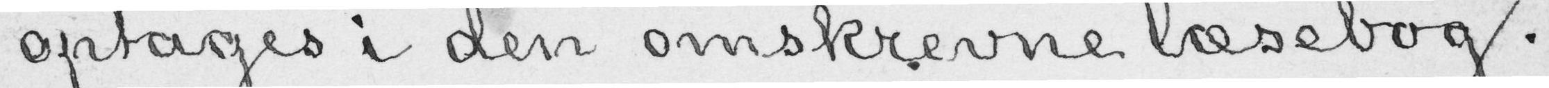optages i den omskrevne læsebog.
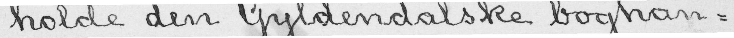holde den Gyldendalske boghan-
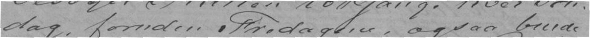dag foruden Fredagene, ogsaa burde
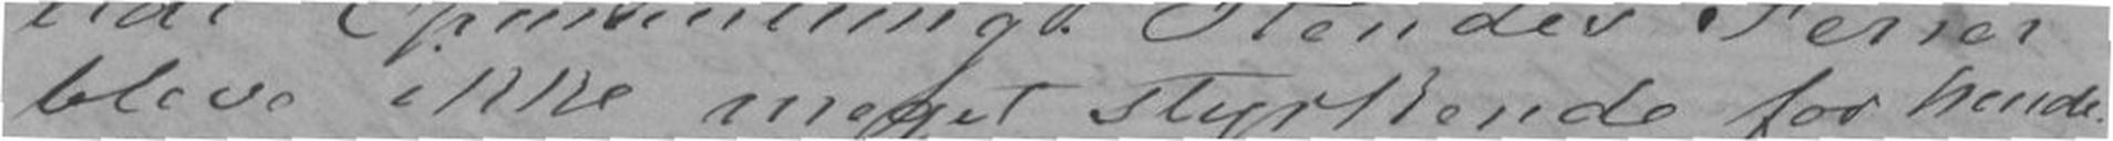bleve ikke meget Styrkende for hende.
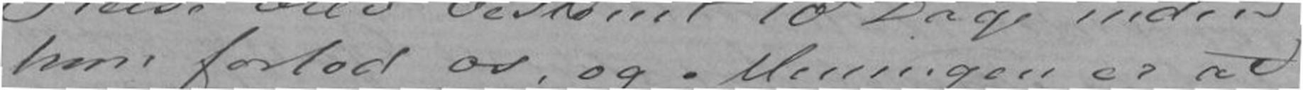hun forlod os, og Meningen er at
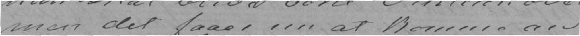men det faaer nu at komme an
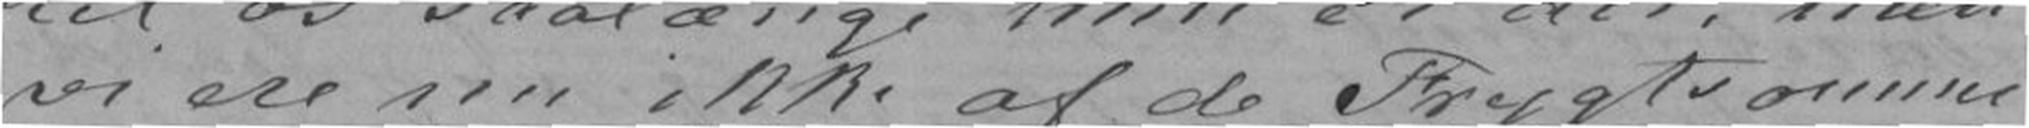vi ere nu ikke af de Frygtsomme
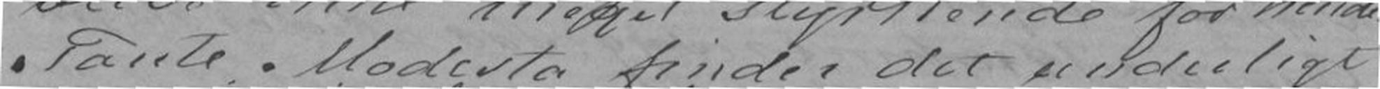Tante Modesta finder det underligt
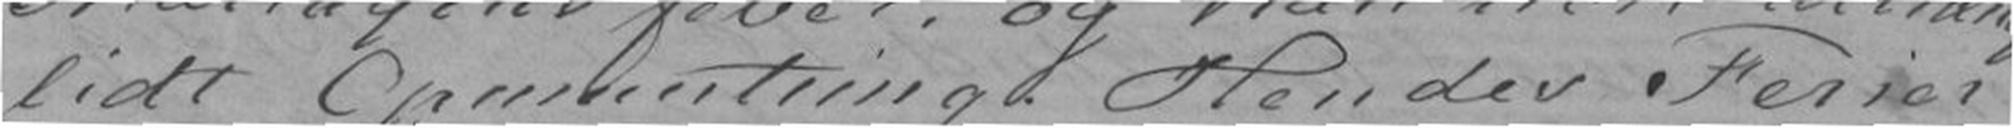lidt Opmuntring. Hendes Ferier
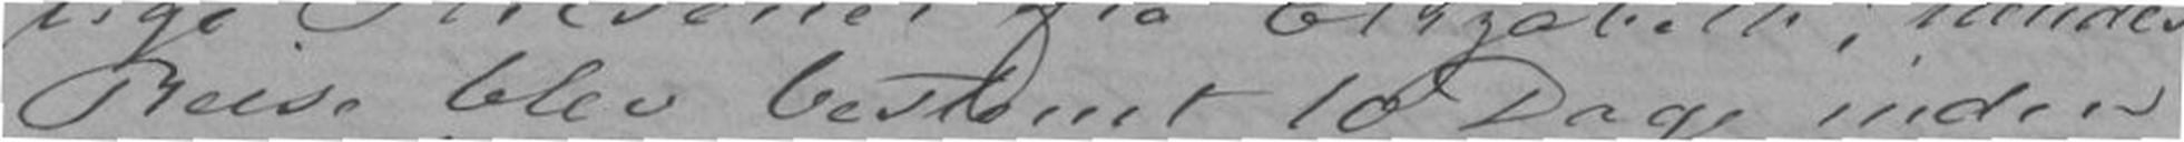Reise blev bestemt 10 Dage inden
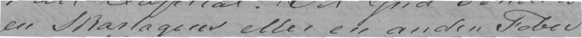en Skarlagens eller en anden Feber
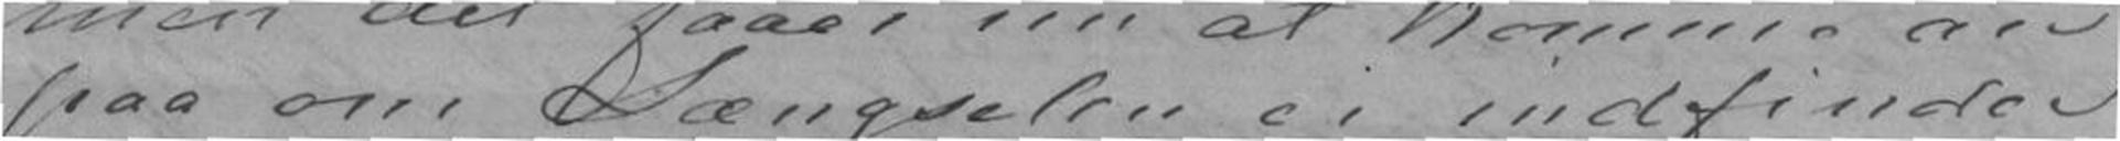paa om Længselen ei indfinder
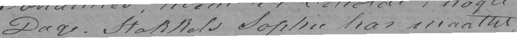Dage. Stakkels Sophie har maattet
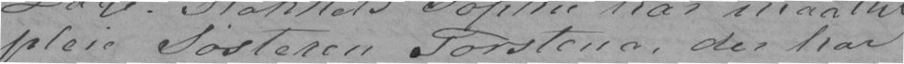pleie Søsteren Torstena, der har
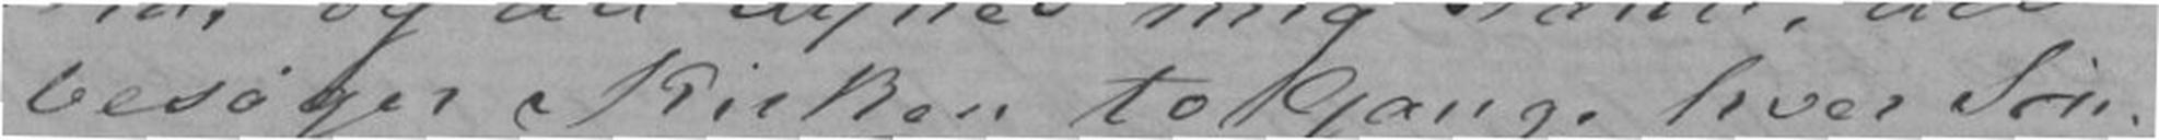besøger Kirken to Gange hver Søn-
